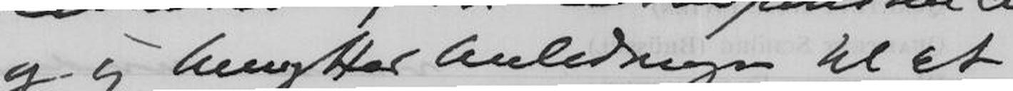og jeg benyttar Anledningen til at
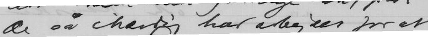De så ihærdig har arbejdet for at
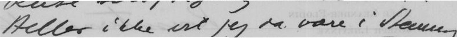Heller ikke vil jeg da være i Stemning
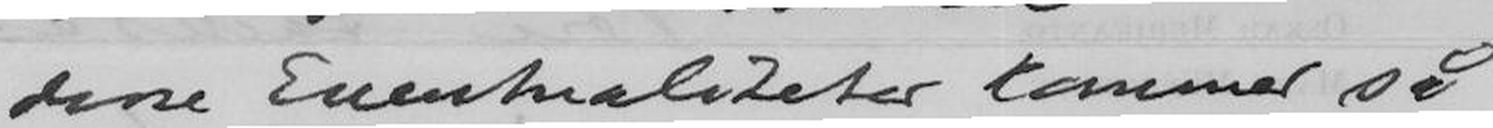disse Eventualiteter kommer så
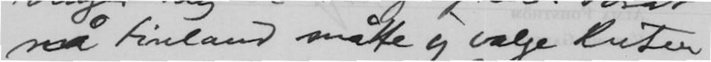nå Finland måtte jeg vælge Ruten
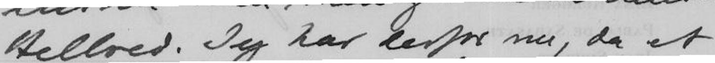Helbred. Jeg har derfor nu, da et
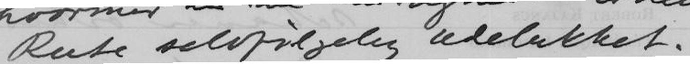Rute selvfølgelig udelukket.
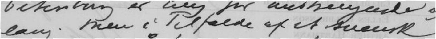lang. Men i Tilfælde af at svensk
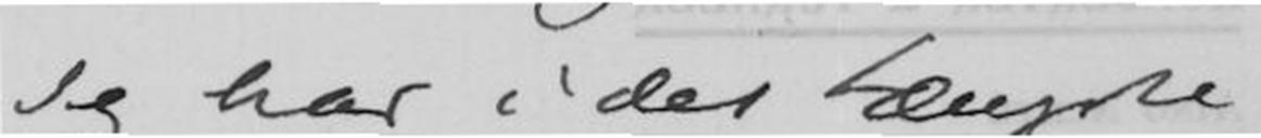Jeg har i det længste
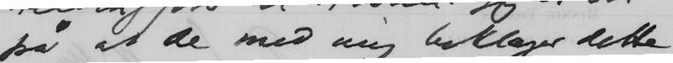på at De med mig beklager dette
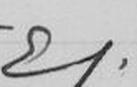E.G.
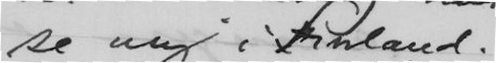se mig i Finland.
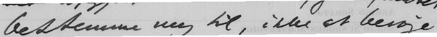bestemme mig til, ikke at besøge
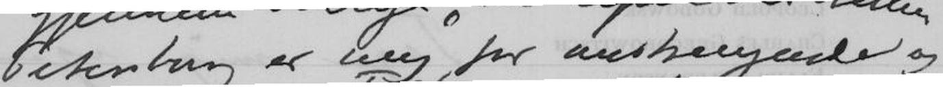Petersburg er mig for anstrengende og
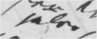selve
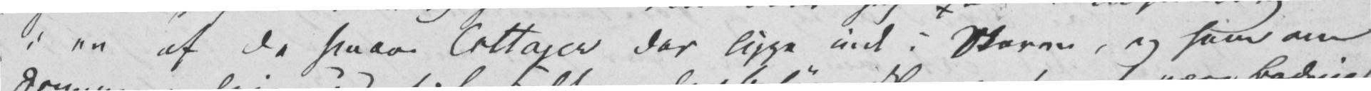i en af de smaae Cottager der ligge inde i Skoven, og som om
